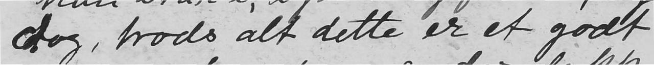dog, trods alt dette er et godt
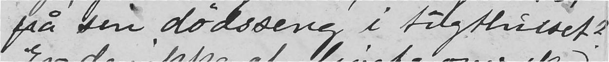på sin dødsseng i tugthuset?
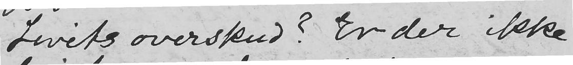Livets overskud? Er der ikke
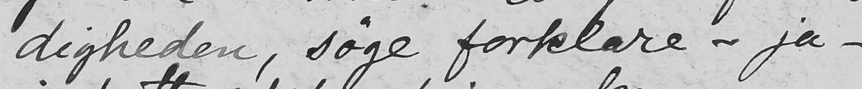digheden, søge forklare - ja -
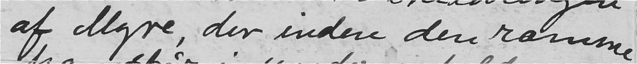af Myre, der inden den ramme
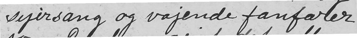sejersang og vajende fanfarer
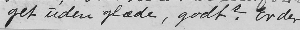get uden glæde, godt? Er der
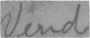Vend
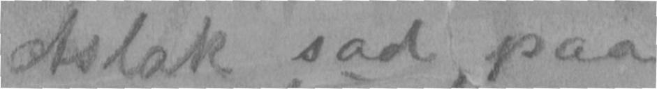Aslak sad paa
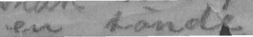en tønde
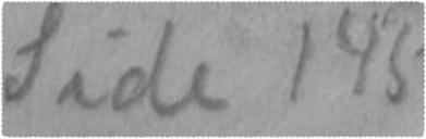Side 143
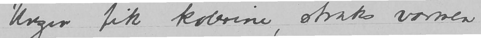Ungen fik kolerine, straks varmen
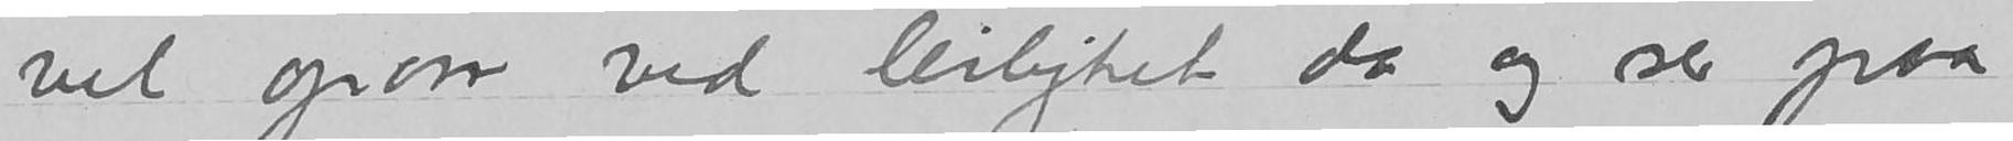vel opom ved leilighet da og ser paa
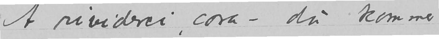A rividerci, cora – du kommer
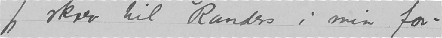Jeg skrev til Randers i min for-
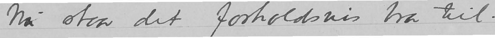Nu staar det forholdsvis bra til.
